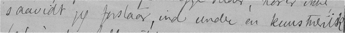saavidt jeg forstaar, ind vnder en kunstnerisk
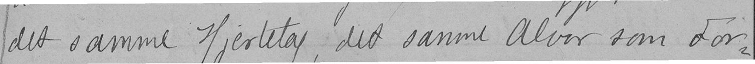det samme Hjertelag, det samme Alvor som For-
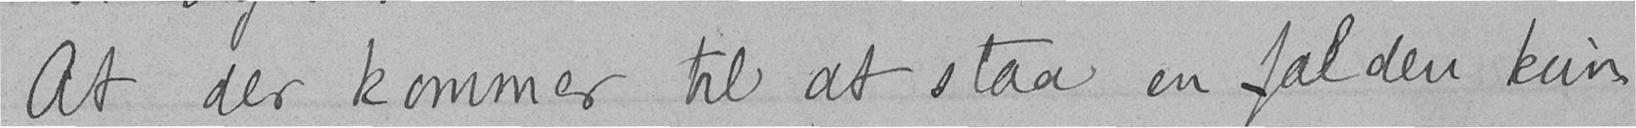At der kommer til at staa en falden kvin-
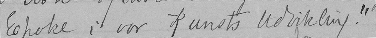Epoke i vor Kunsts Udvikling."
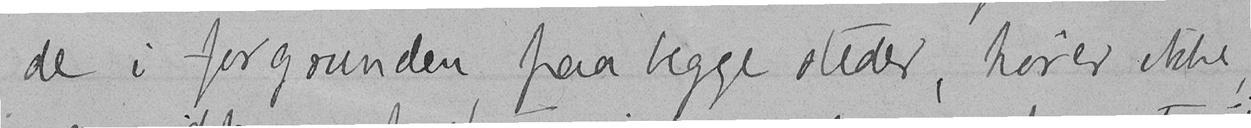de i forgrunden paa begge steder, hører ikke,
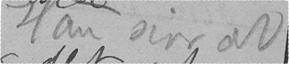Han siger at
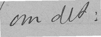om det:
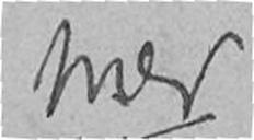mer
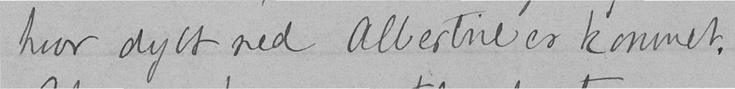hvor dybt ned Albertine er kommet.
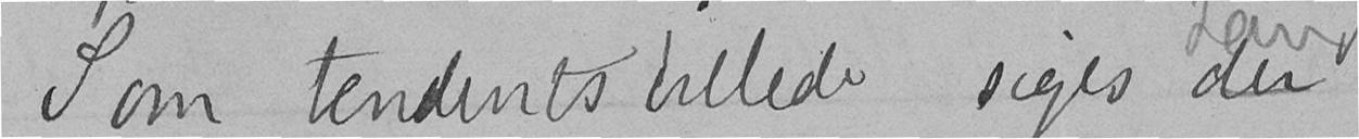Som tendentsbillede, siges der
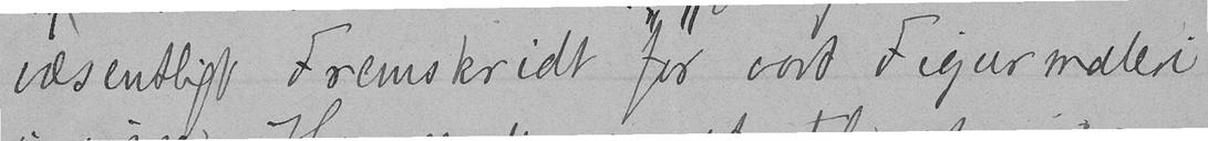væsentligt Fremskridt for vort Figurmaleri
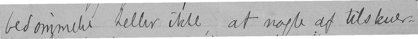bedømmelse heller ikke, at nogle af tilskuer-
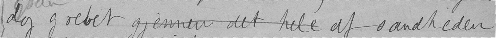dog grebet gjennem det hele af sandheden
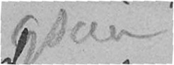ogsaa
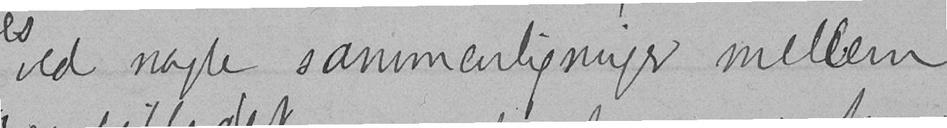ved nogle sammenligninger mellem
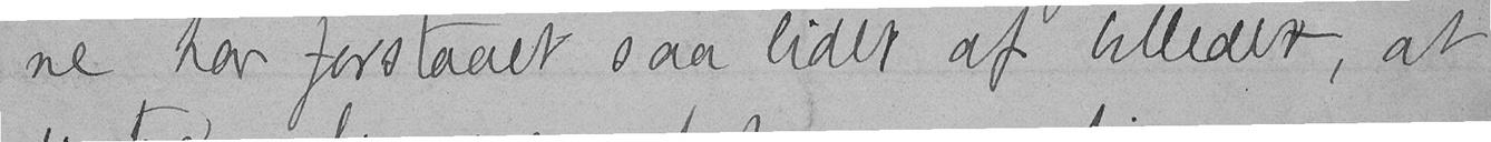ne har forstaaet saa lidet af billedet, at
